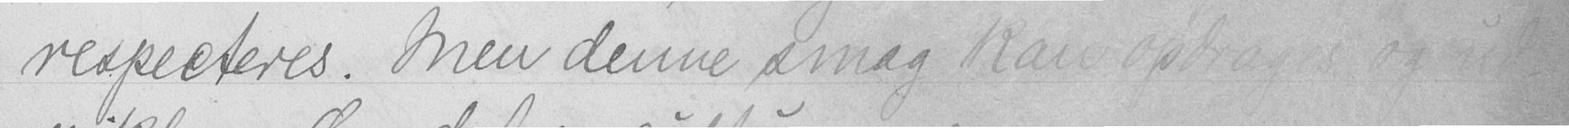respecteres. Men denne smag kan opdrages og ud-
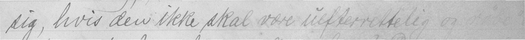sig, hvis den ikke skal være uefterrettelig og "røbe
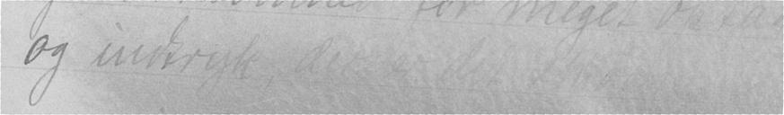og indtryk, der er det
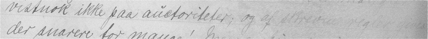vistnok ikke paa autoriteter; og af skrevne regler gives
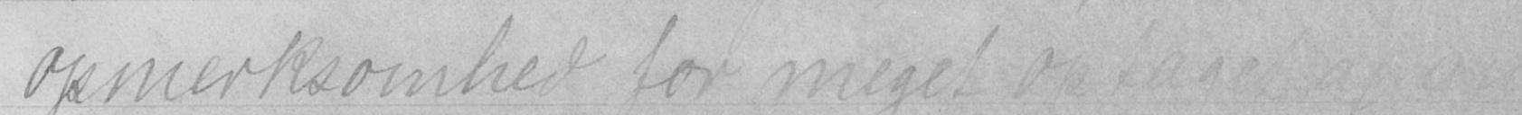opmerksomhed for meget optage
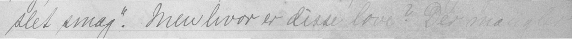slet smag." Men hvor er disse love? Der mangler
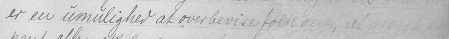er en umulighed at overbevise folk om, at noget er
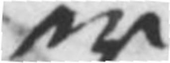er
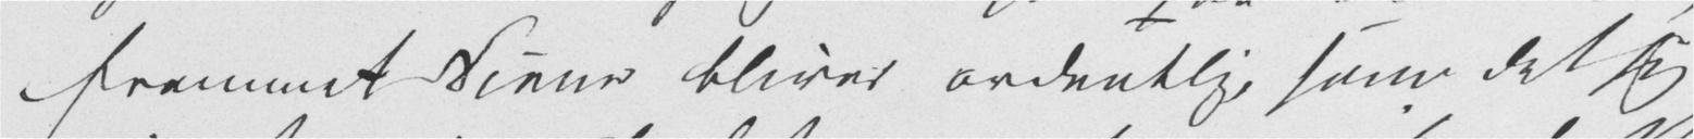fremmet Scene bliver ordentlig, som det sig
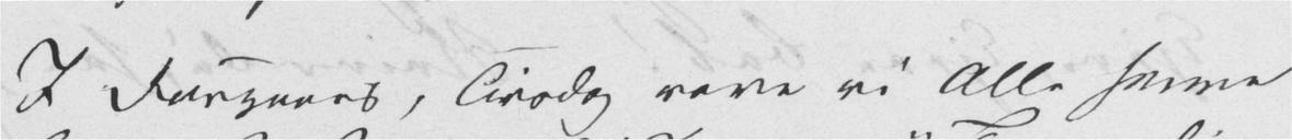I Forgaars, Tirsdag vare vi Alle henne
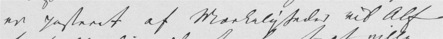er passeret af Mærkeligheder vil Alf
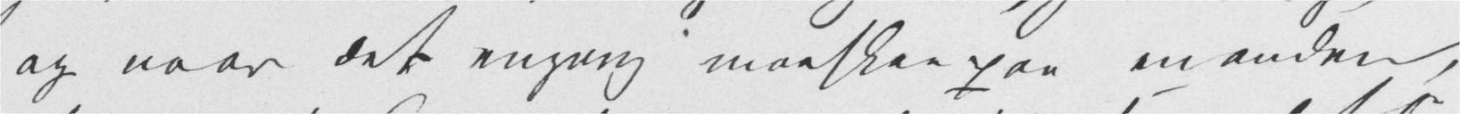og naar det engang maaske paa en anden,
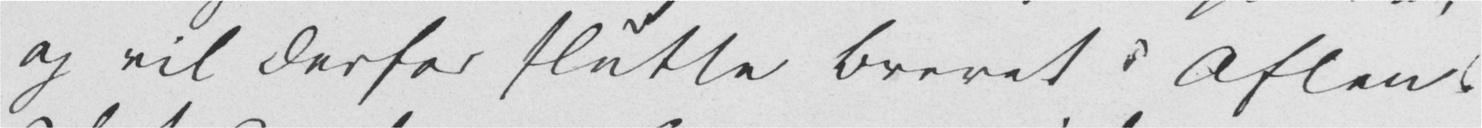og vil derfor slutte Brevet i Aften.
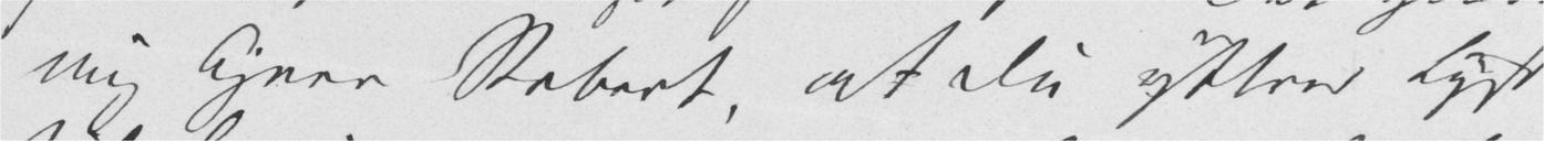mig kjere Robert, at Du yttrer Lyst
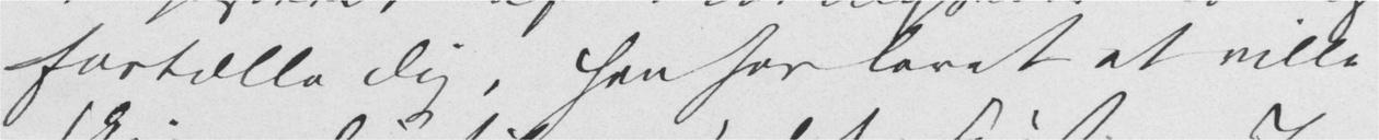fortælle Dig, han har lovet at ville
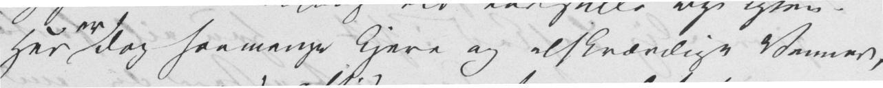Her er dog saa mange Kjere og elskværdige Venner,
